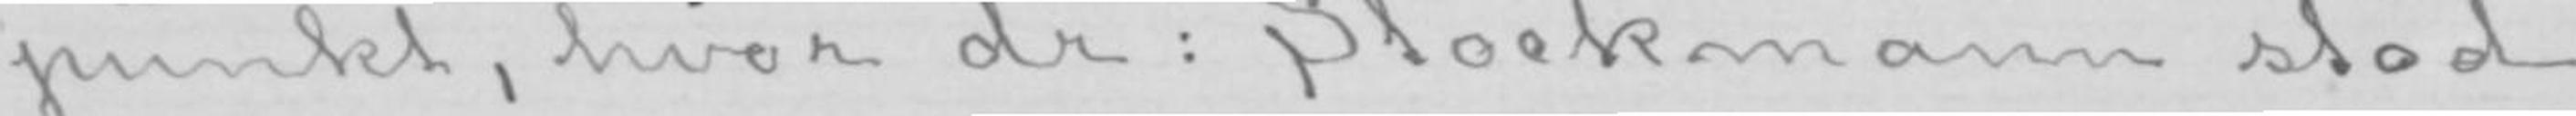punkt, hvor dr: Stockmann stod
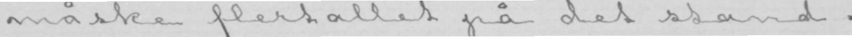måske flertallet på det stand-
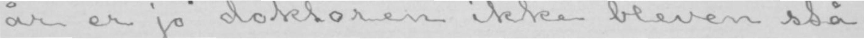år er jo doktoren ikke bleven stå-
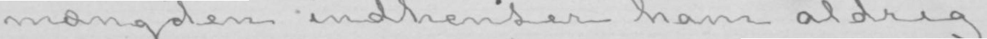mængden indhenter ham aldrig;
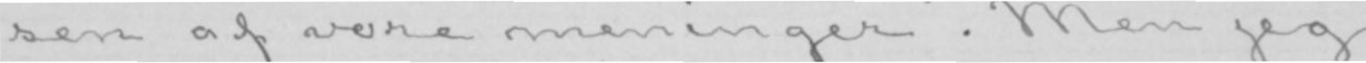sen af vore meninger. Men jeg
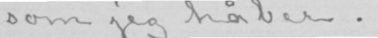som jeg håber.-
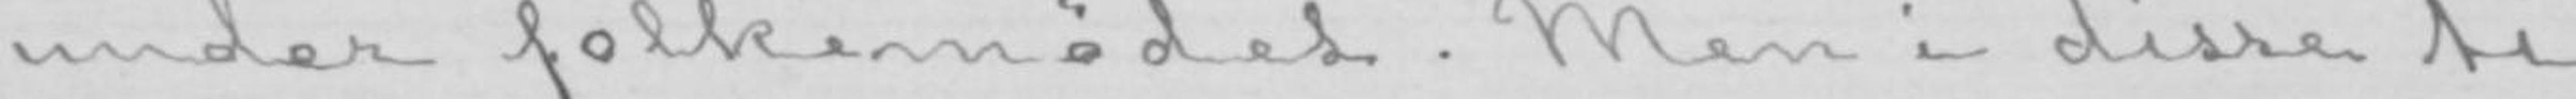under folkemødet. Men i disse ti
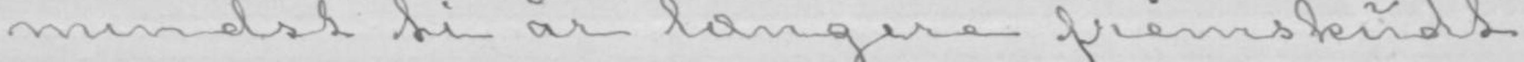mindst ti år længere fremskudt
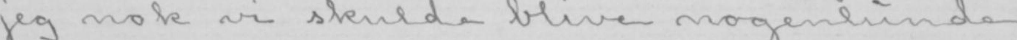jeg nok vi skulde blive nogenlunde
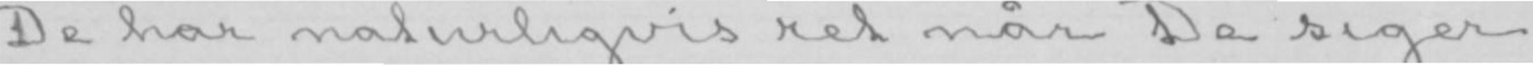De har naturligvis ret når De siger
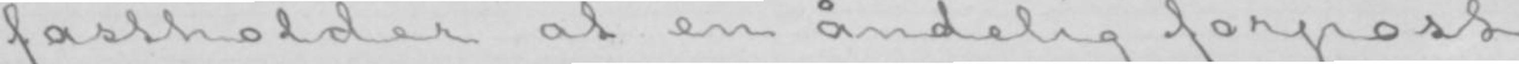fastholder at en åndelig forpost-
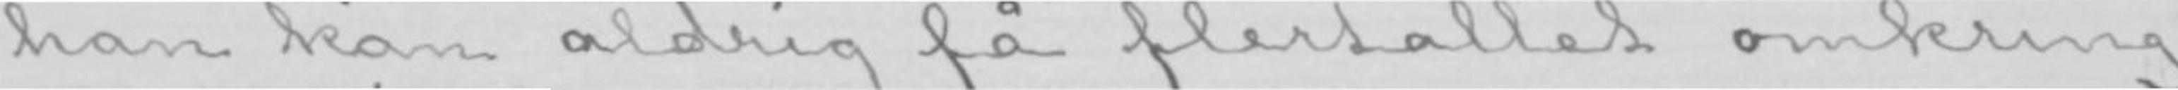han kan aldrig få flertallet omkring
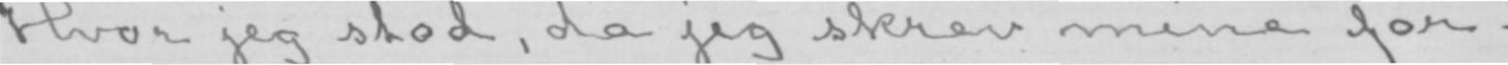Hvor jeg stod, da jeg skrev mine for-
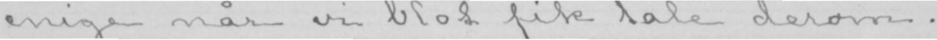enige når vi blot fik tale derom.
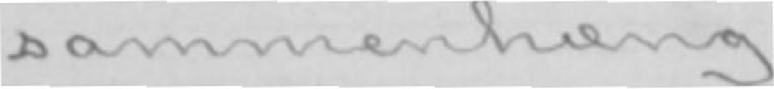sammenhæng.
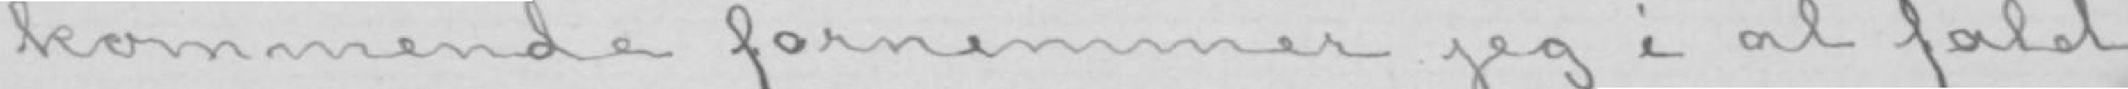kommende fornemmer jeg i al fald
